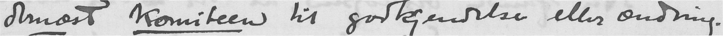dernæst komiteen til godkjendelse eller ændring.
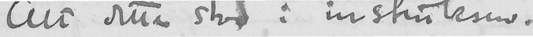Alt dette stod i indstruksen.
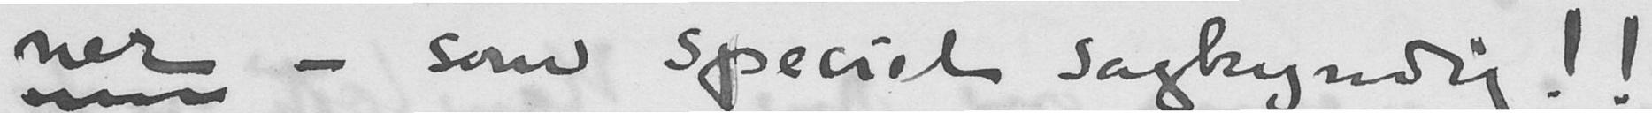ner - som speciel sagkyndig!!
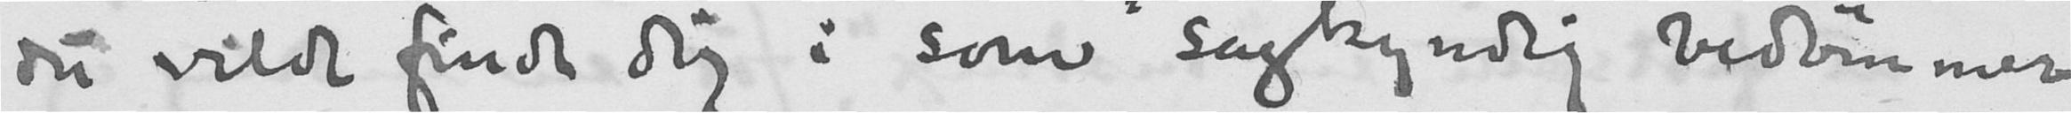du vilde finde dig i som "sagkyndig" bedømmer
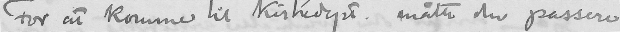For at komme til kirkedept. måtte den passere
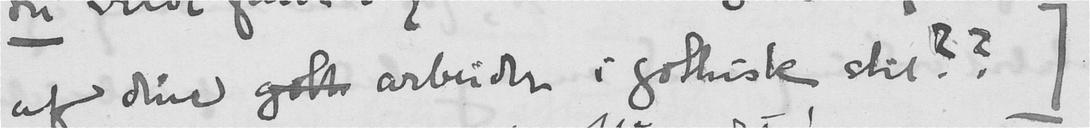af dine goth arbeider i gothisk stil?? ]
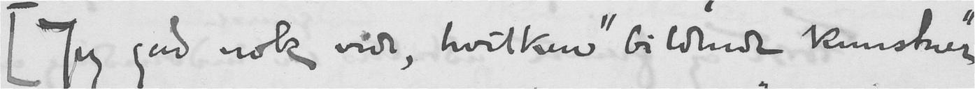[ Jeg gad nok vide, hvilken "bildende kunstner"
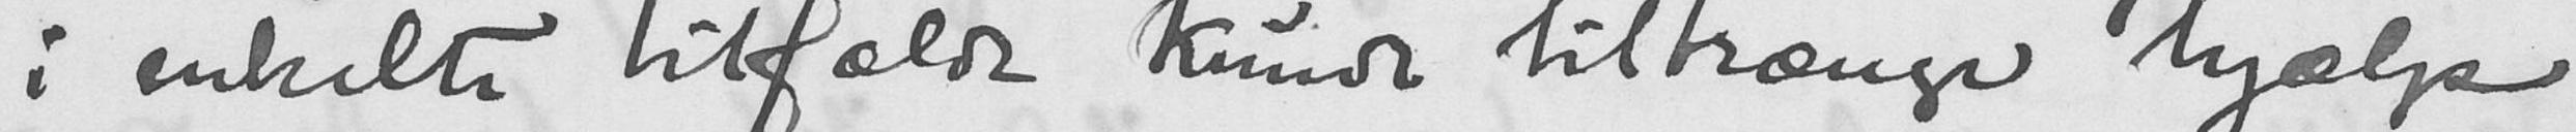i enkelte tilfælde kunde tiltrænge hjælp
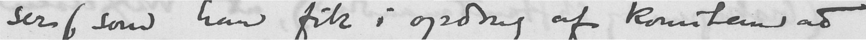ser, (som han fik i opdrag af komiteen at
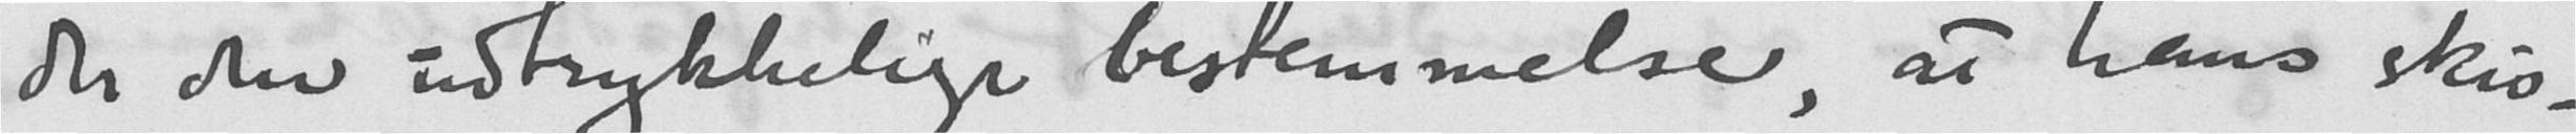der den udtrykkelige bestemmelse, at hans skis-
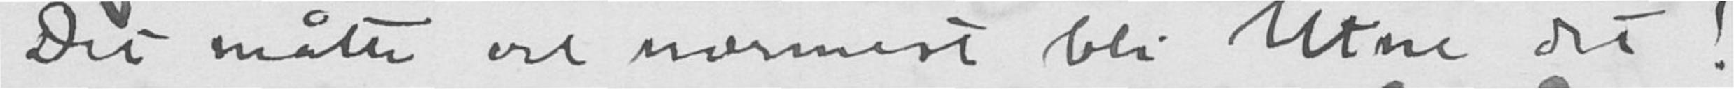Det måtte vel nærmest bli Utne det!
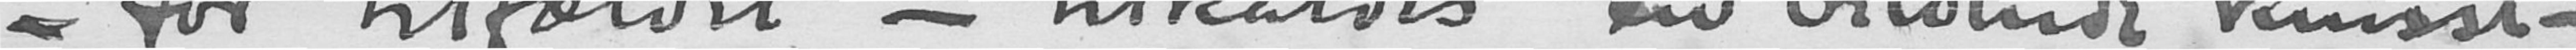- for tilfældet - tilkaldes en bildende kunst-
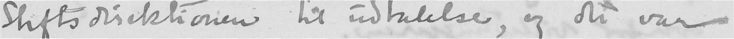stiftsdirektionen til udtalelse, og det var
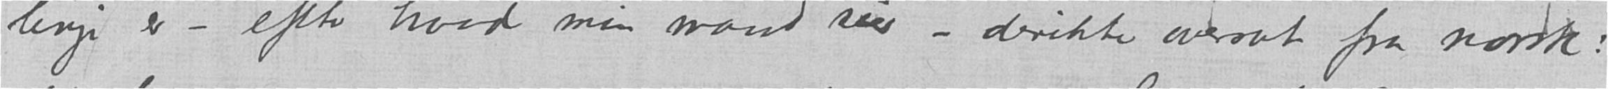linje er – efter hvad min mand sier – direkte oversat fra norsk:
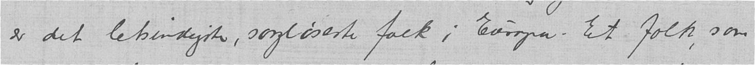er det letsindigste, sorgløseste folk i Europa. Et folk, som
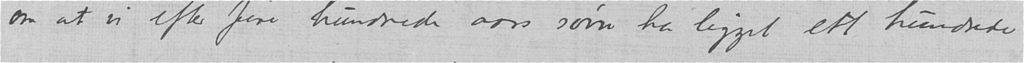om at vi efter fire hundrede aars søvn har ligget ett hundrede
